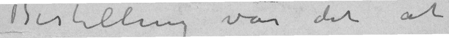Bestilling var det at
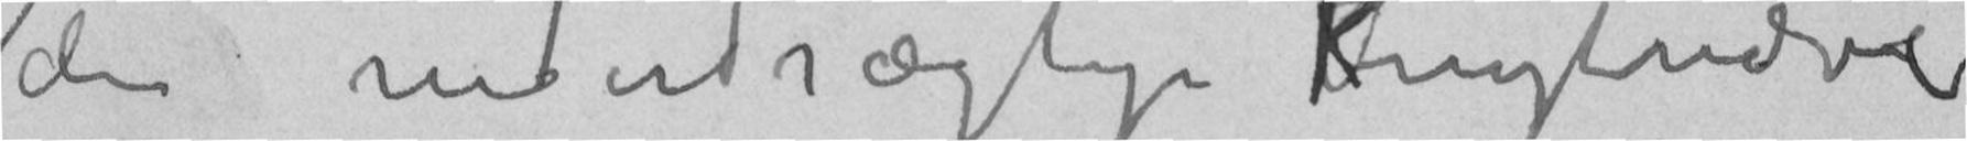og den nederdrægtige Knytnæve
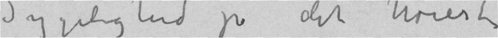Sygelighed på det høieste
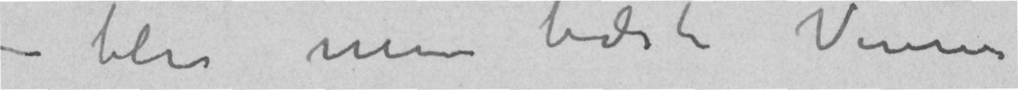– blev mine bedste Venner
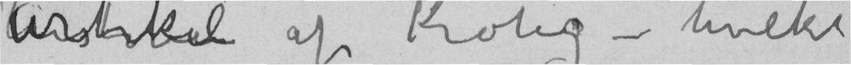artikel af Krohg – hvilket
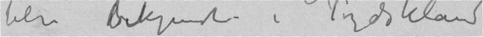ble bekjent i Tydskland
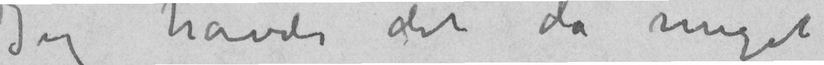Jeg havde det da meget
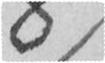8)
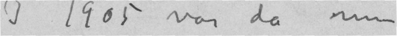I 1905 var da min
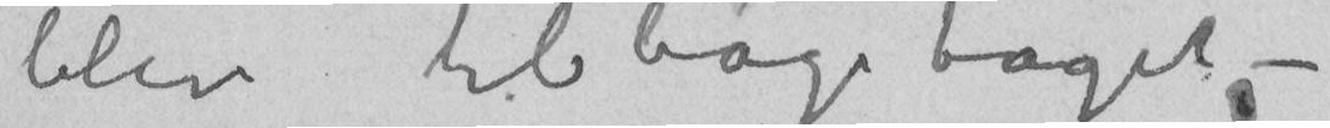blev tilbagetaget –
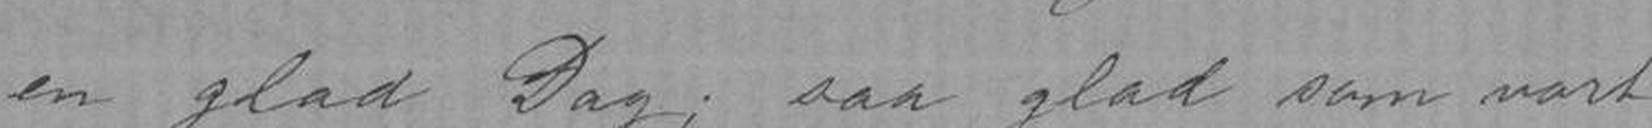en glad Dag; saa glad som vort
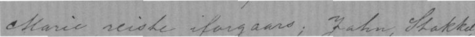Marie reiste iforgaars; John, Stakkel,
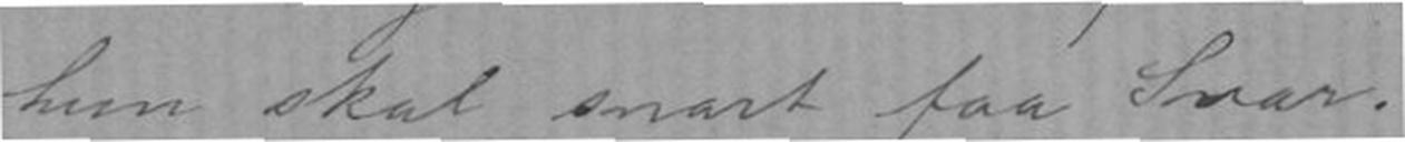hun skal snart faa Svar.
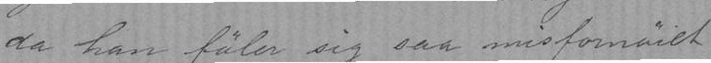da han føler sig saa misfornøiet
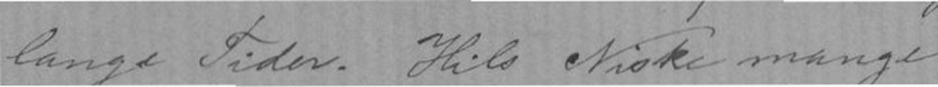lange Tider. Hils Niske mange
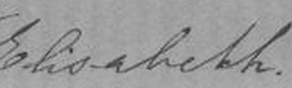Elisabeth.
